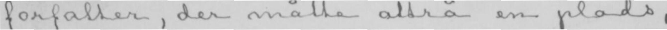forfatter, der måtte attrå en plads,
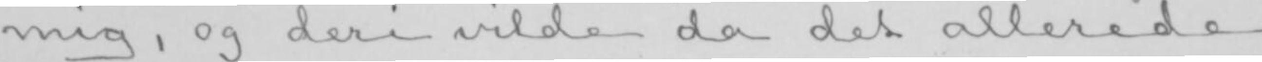mig, og deri vilde da det allerede
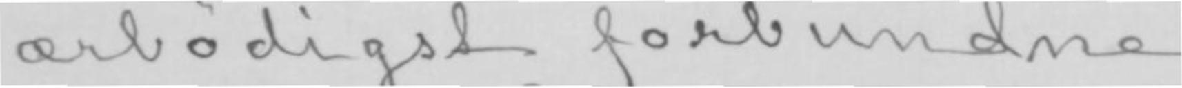ærbødigst forbundne
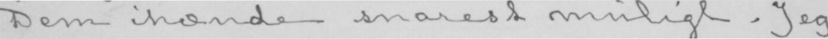Dem ihænde snarest muligt. Jeg
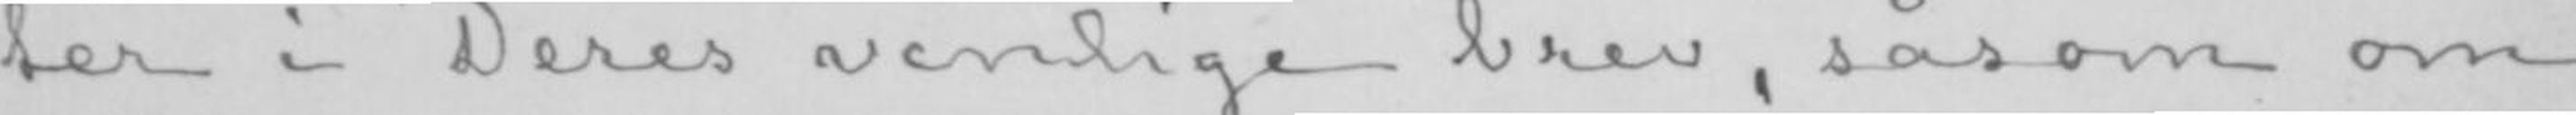ter i Deres venlige brev, såsom om
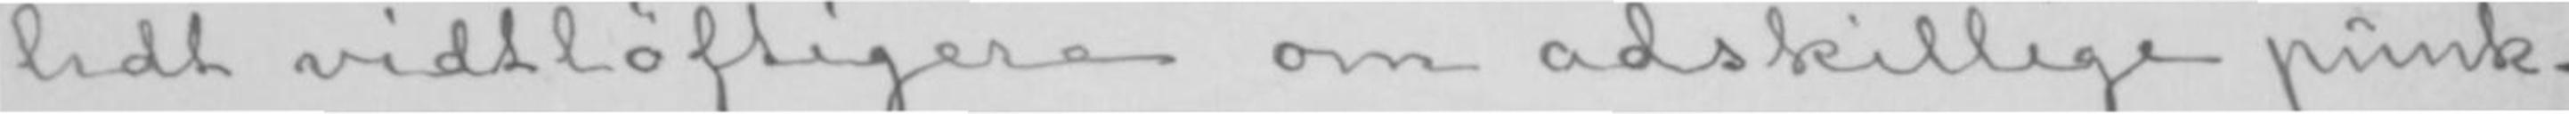lidt vidtløftigere om adskillige punk-
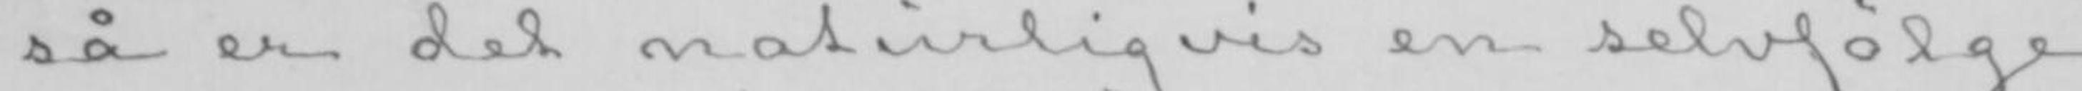så er det naturligvis en selvfølge
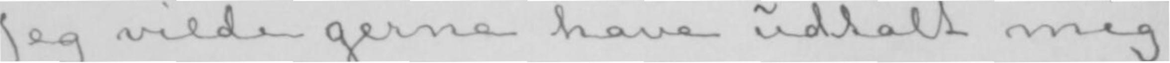Jeg vilde gerne have udtalt mig
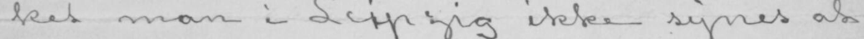ket man i Leipzig ikke synes at
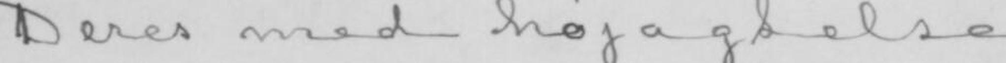Deres med højagtelse
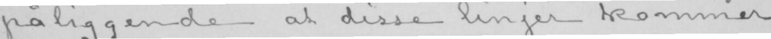påliggende at disse linjer kommer
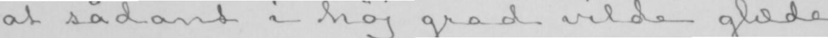at sådant i høj grad vilde glæde
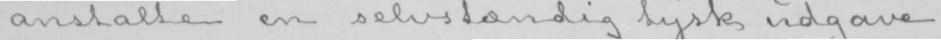anstalte en selvstændig tysk udgave
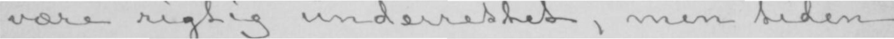være rigtig underrettet, men tiden
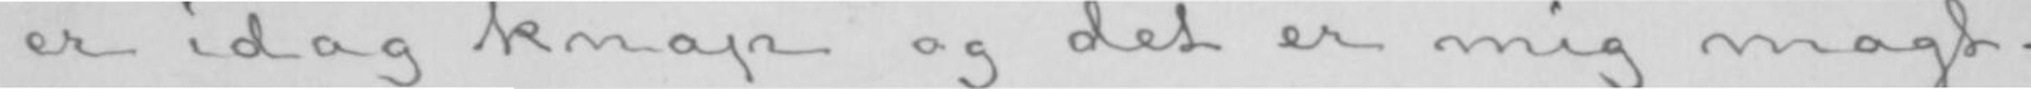er idag knap og det er mig magt-
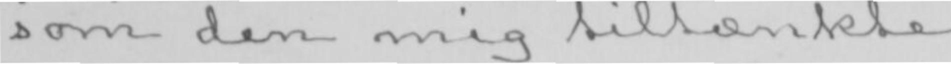som den mig tiltænkte.
